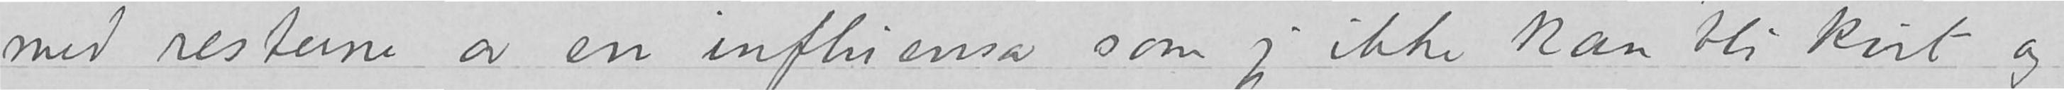med resterne av en influensa som jeg ikke kan bli kvit og
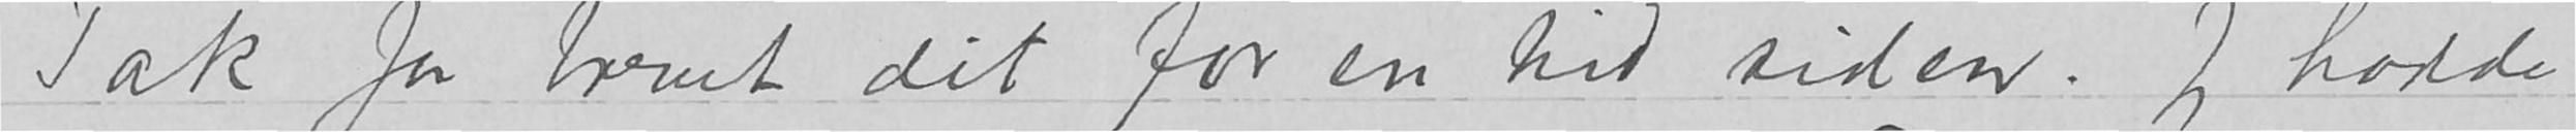Tak for brevet dit for en tid siden. Jeg hadde
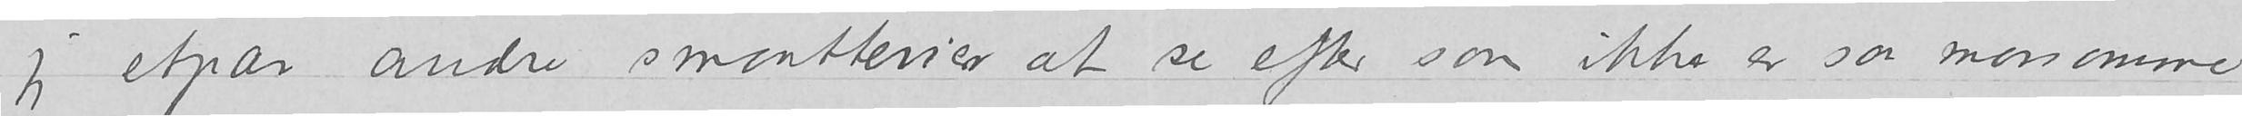jeg etpar andre smaatterier at se efter som ikke er saa morsomme
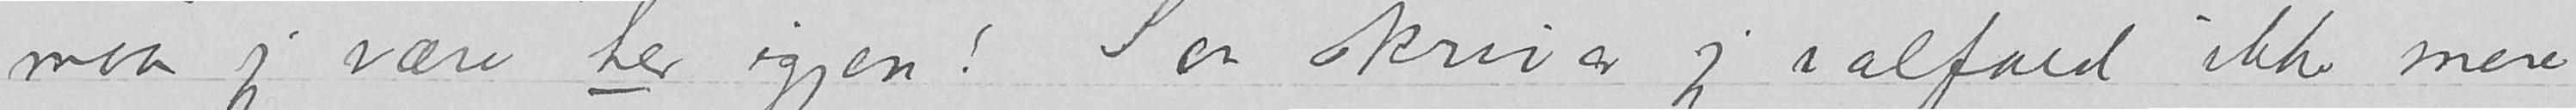maa jeg være her igjen! Saa skriver jeg ialfald ikke mere
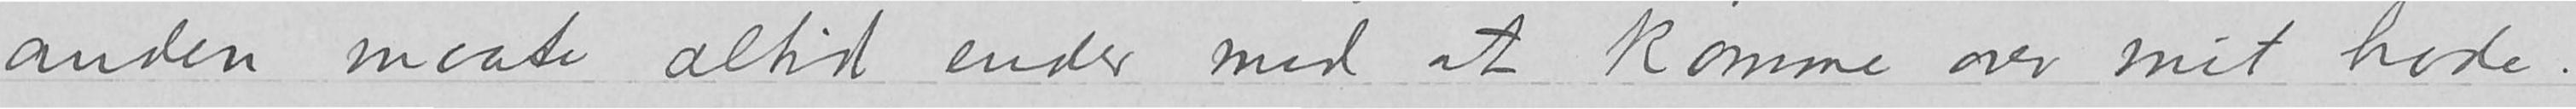anden maate altid ender med at komme over mit hode.
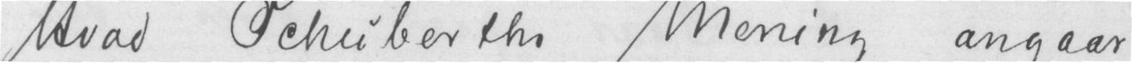Hvad Schuberths Mening angaar,
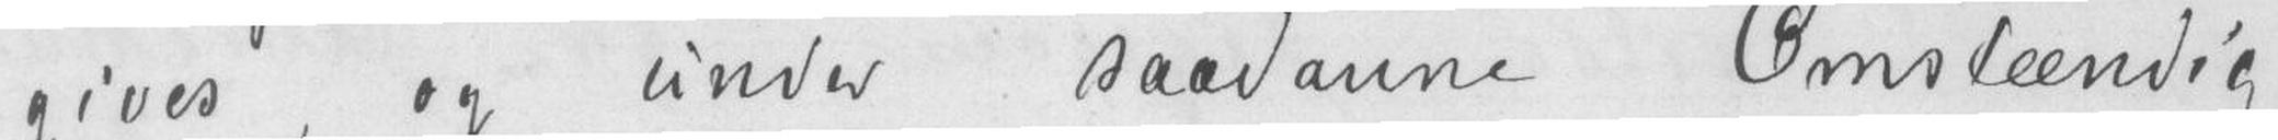gives, og under saadanne Omsteendig-
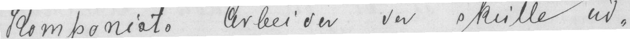Komponisto Arbeider der skulle ud-
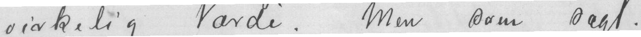virkelig Værdi. Men som sagt,
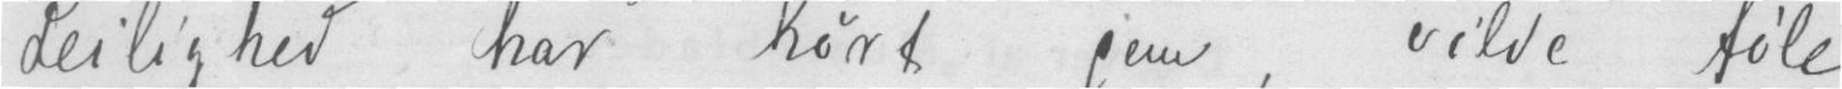Leilighed har hørt dem, vilde føle
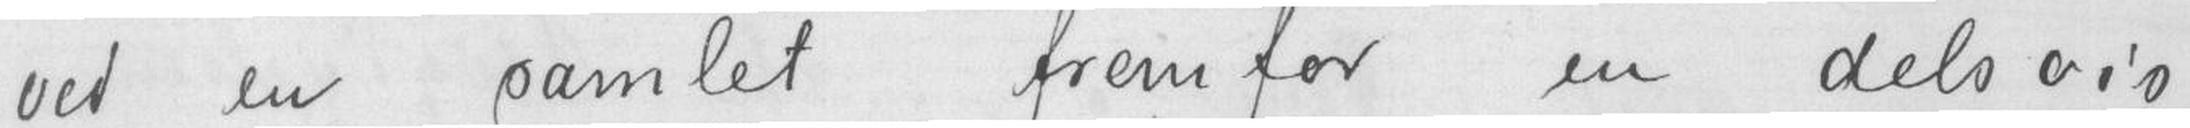ved en samlet frefor en delsvis
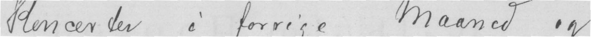Koncerter i forrige Maaned og
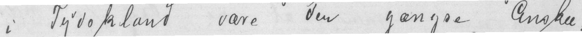i Tydskland være den gængse Ansku-
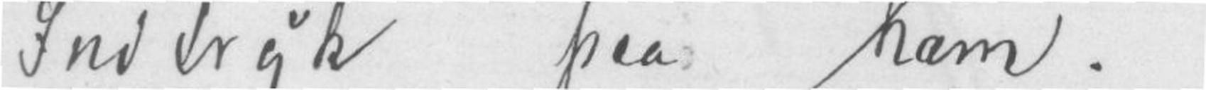Indtryk paa ham.
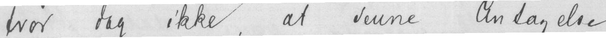tror dog ikke, at denne Antagelse
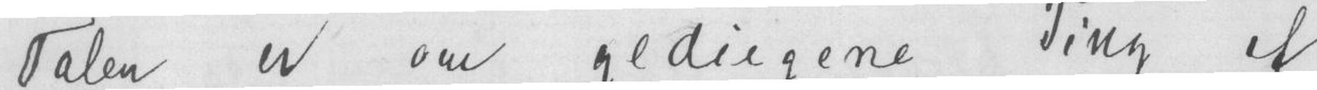Talen er om gediegene Ting af
